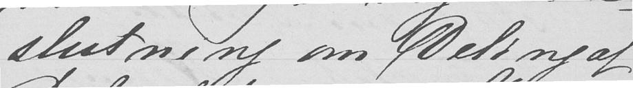slutning om Deling af
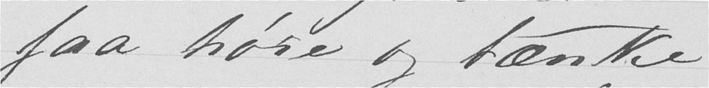faa høre og tænke
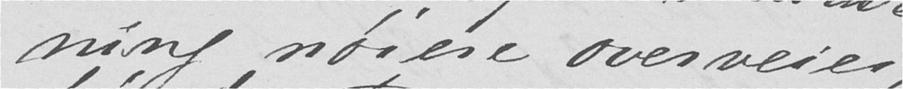ning nøiere overveies,
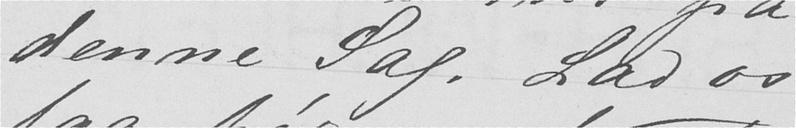denne Sag. Lad os
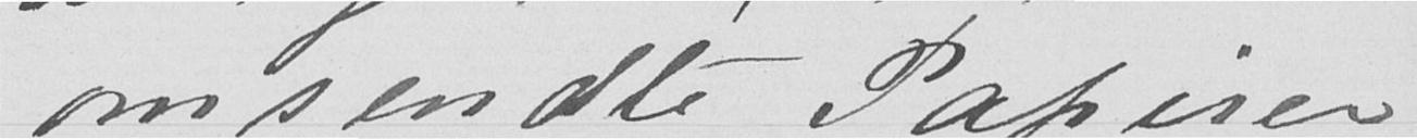omsendte Papirer
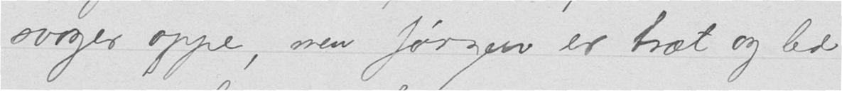svoger oppe, men Jørgen er træt og led
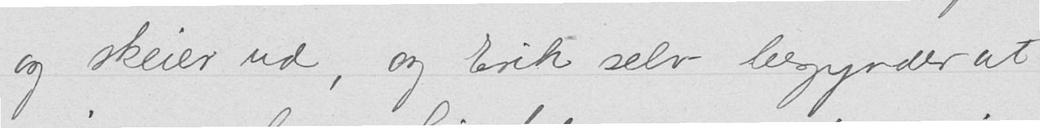og skeier ud, og Erik selv begynder at
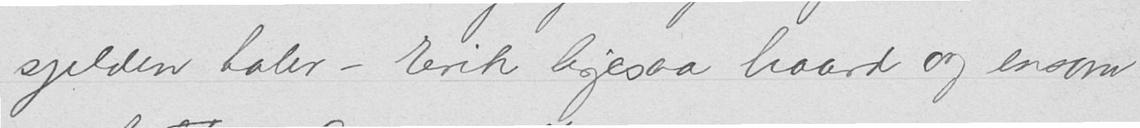sjelden taler - Erik ligesaa haard og ensom
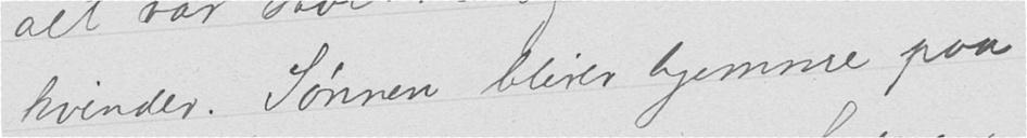kvinder. Sønnen bliver hjemme paa
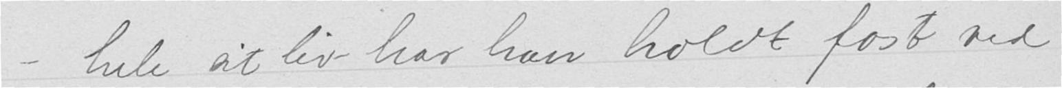- hele sit liv har han holdt fast ved
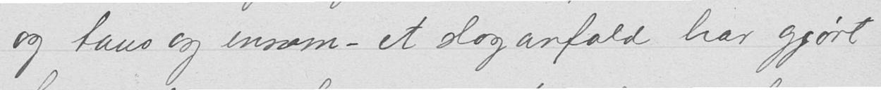og taus og ensom - et slaganfald har gjort
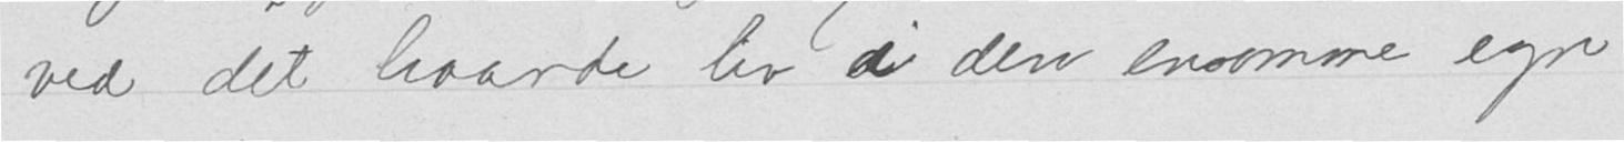ved det haarde ler i den ensomme egn
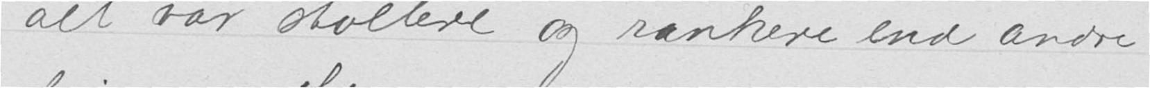alt var stoltere og rankere end andre
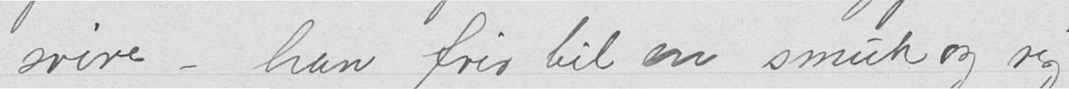svire – han frir til en smuk og rig
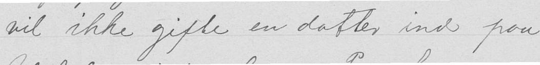vil ikke gifte en datter ind paa
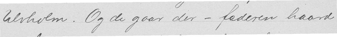Ulvholm. Og de gaar der - faderen haard
